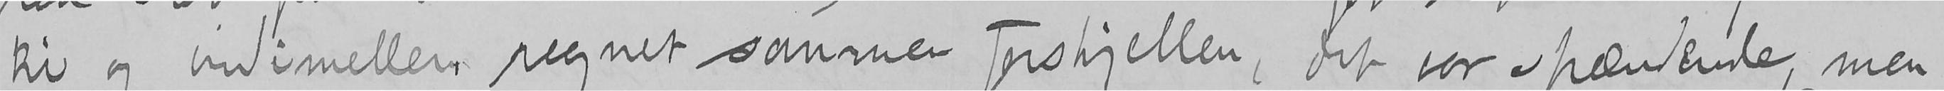ki og indimellem regnet sammen forskjellen, det var spændende, men
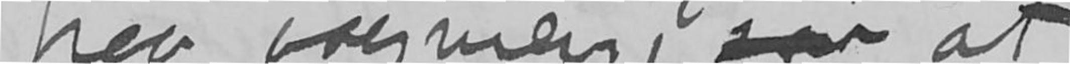paa vognen, saa at
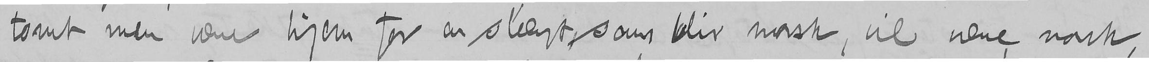tomt men være hjem for en slægt som blir norsk, vil være norsk,
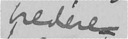hedrere
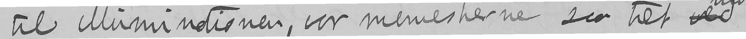til illuminationen, var menneskerne saa tæt ved
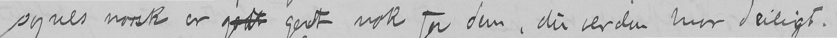synes norsk er godt godt nok for dem, du verden hvor deiligt.
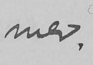mer.
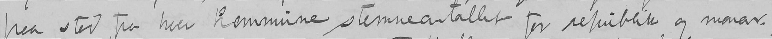paa stod fra hver kommune stemmeantallet for republik og monar-
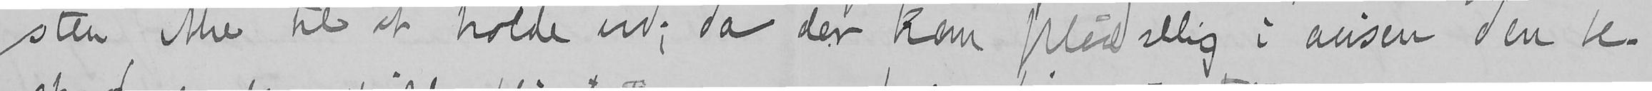sten ikke til at holde ud; da der kom pludselig i avisen den be-
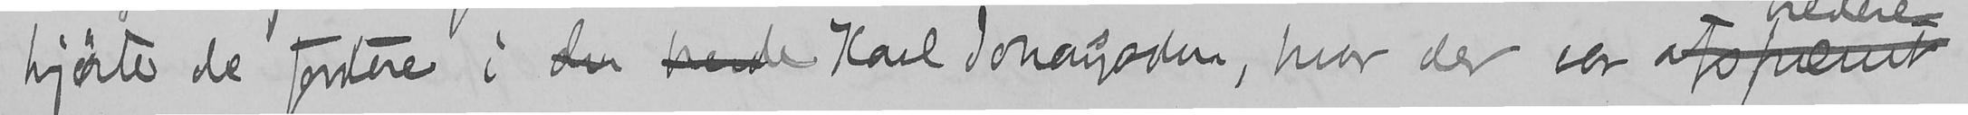kjørte de fortere i den havde Karl Johansgaden, hvor der var afsperret
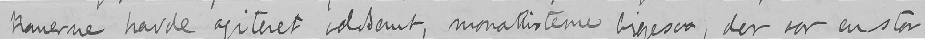kanerne havde agiteret voldsomt, monarkisterne ligesaa, der var en stor
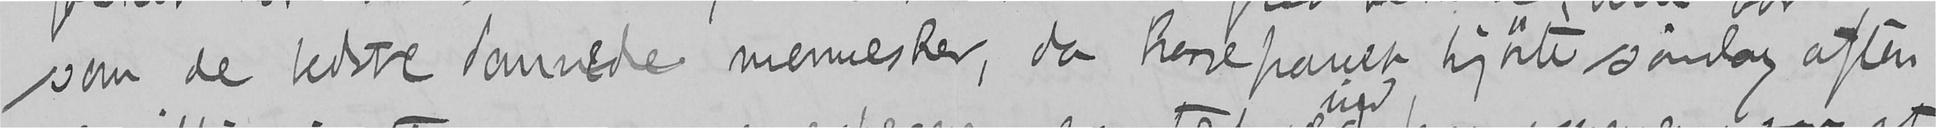som de bedste dannede mennesker, da kongeparret kjørte søndag aften
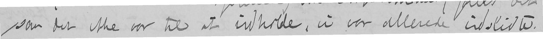som det ikke var til at udholde, vi var allerede udslidte.
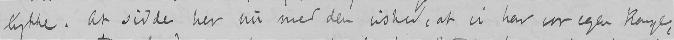lykke. At sidde her nu med den vished, at vi har vor egen konge,
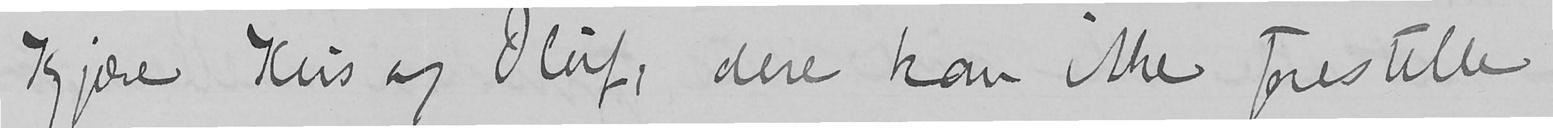Kjære Kris og Oluf, dere kan ikke forestille
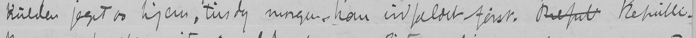kulden jaget os hjem, tirsdag morgen kom udfaldet først. Reepub Republi-
